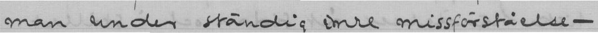man under ständig inre missförståelse -
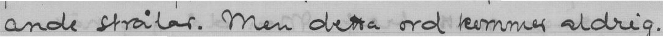ande strålar. Men detta ord kommer aldrig.
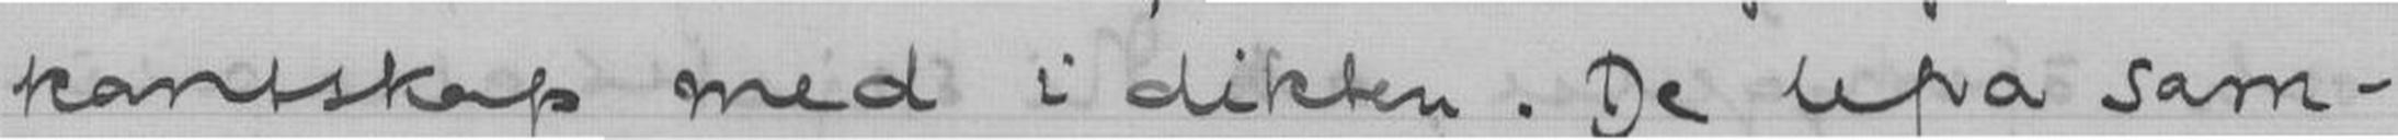kantskap med i dikten. De lefva sam-
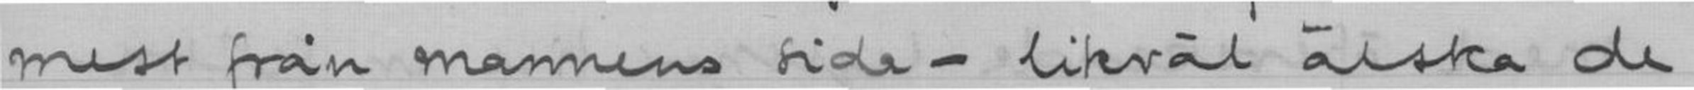mest från mannens side - likväl älska de
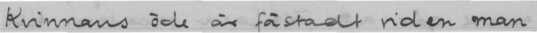Kvinnans öde är förstadt vid en man,
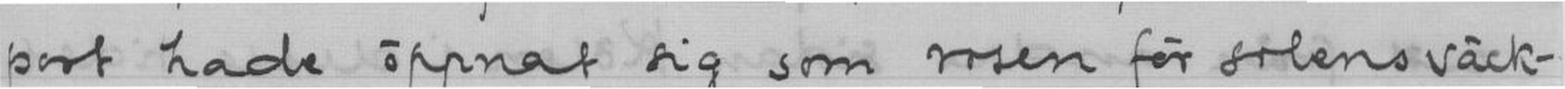port hade öppnat sig som rosen för solens väck-
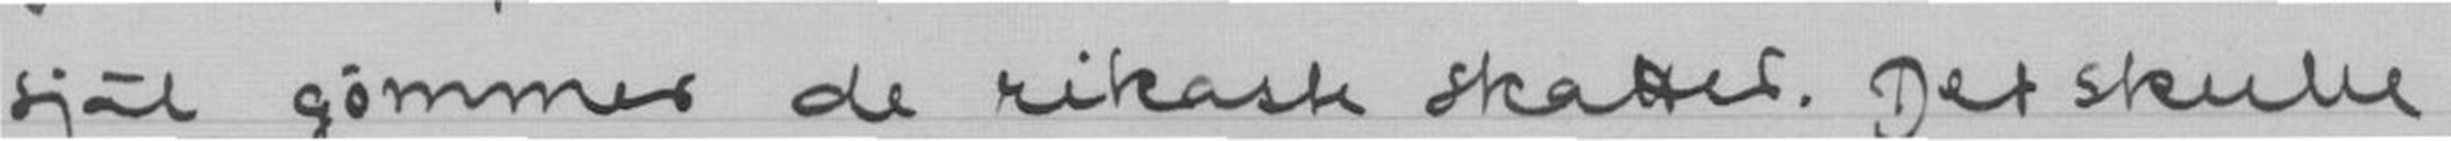själ gömmer de rikaste skatter. Det skulle
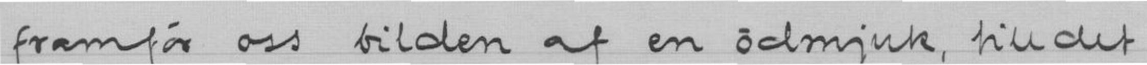framför oss bilden af en ödmjuk, till det
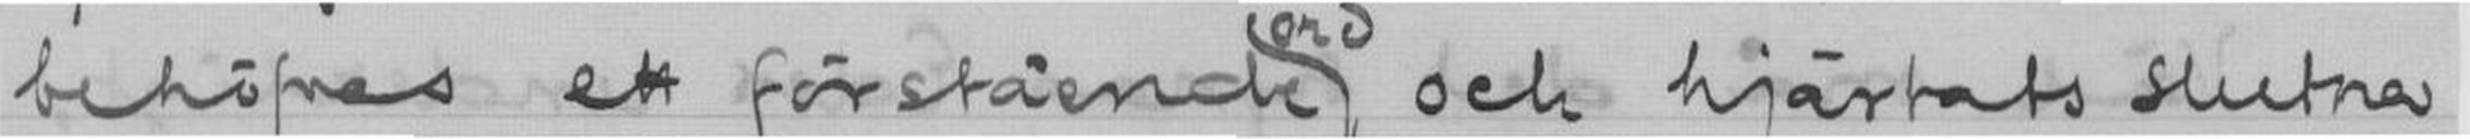behöfvas ett förstående ord och hjärtats slutna
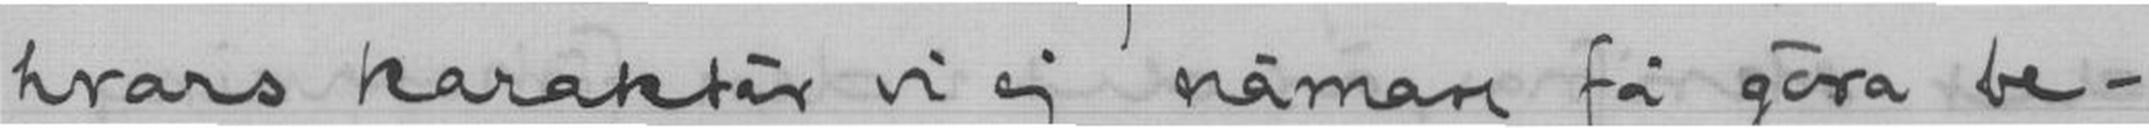hvars karaktär vi ej nämare få göra be.
